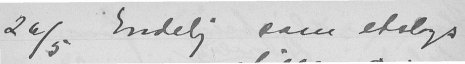26/5 Endelig som etslags
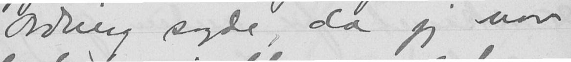Ording sagde, da jeg var
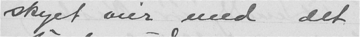skyet veir med det
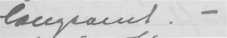langsomt. -
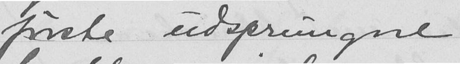første udsprungne
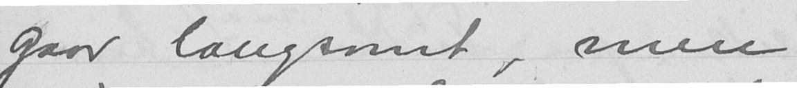gaar langsomt, men
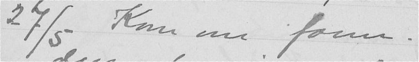27/5 Kom om form.
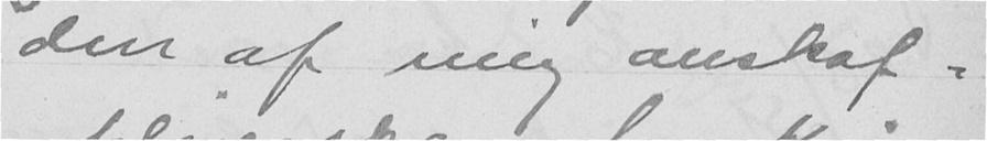den af mig anskaf-
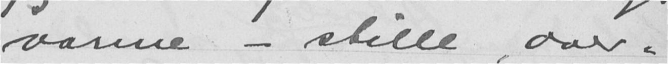varme - stille, over-
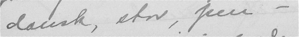dansk, stor, pen -
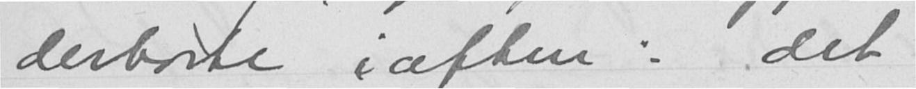derborte i aften: det
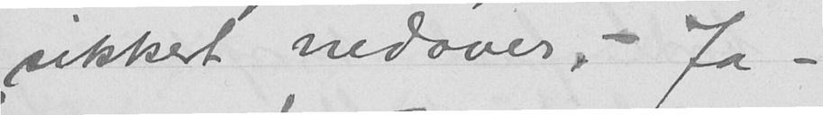sikkert nedover, - Ja -
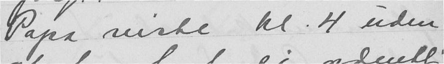Papa reiste kl. 4 uden
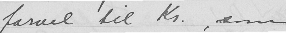farvel til Kr., som
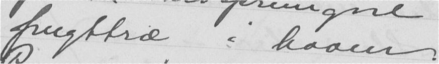frugttræ i haven.
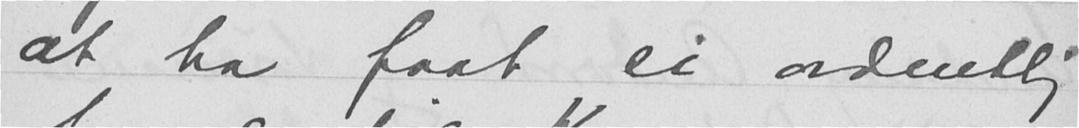at ha faat ei ordentlig
